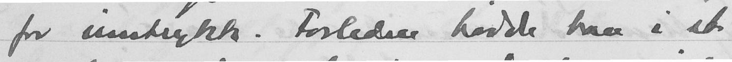for inntrykk. Forleden hadde han i et
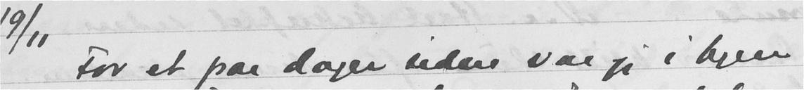19/11 For et par dager siden var jeg i byen
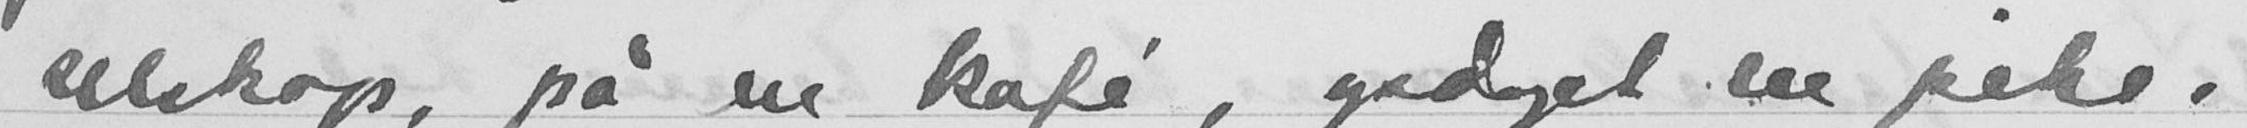selskap, på en kafé, opdaget en pike,
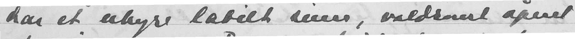har et uhyre labilt sinn, voldsomt åpent
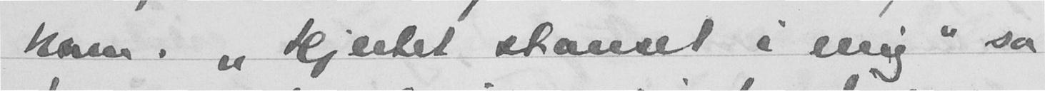ham. "Hjertet stanset i mig" sa
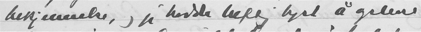bekjennelse, og jeg hadde heftig lyst å opleve
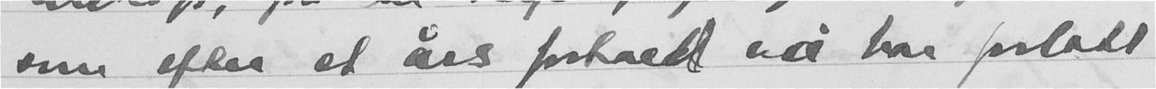som efter et års forhold nå har forlatt
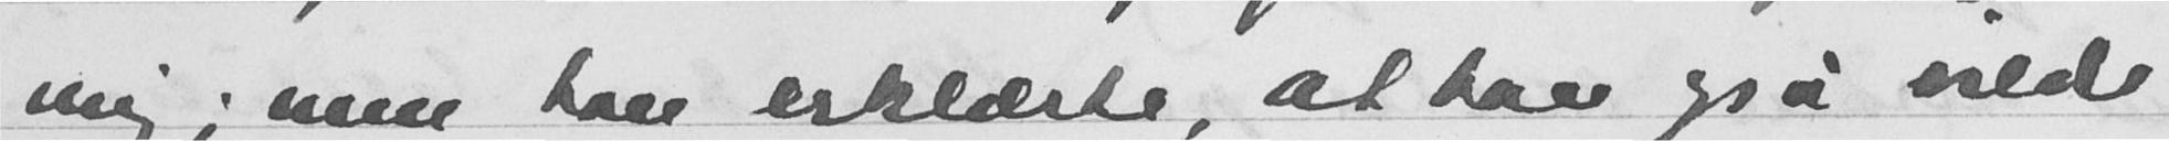mig; men han erklærte, at han også vilde
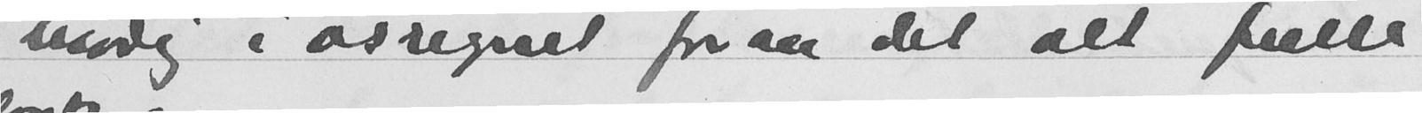modig i øsregnet foran det alt fulle
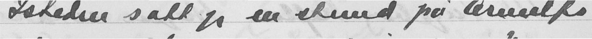Isteden satt jeg en stund på Arnulfs
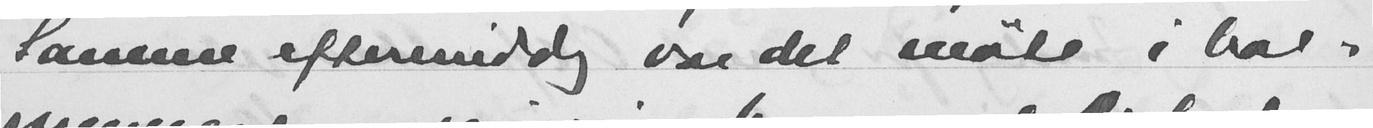Samme eftermiddag var det møte i bal-
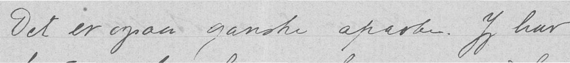Det er også ganske aparte. Jeg har
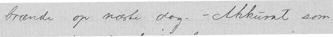brænde op næste dag. - Akkurat som
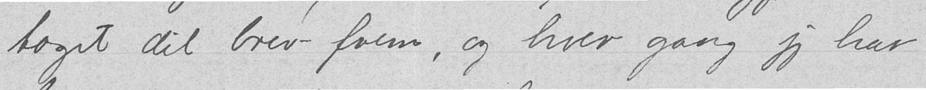taget dit brev frem, og hver gang jeg har
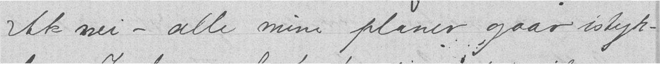Akk nei - alle mine planer gaar istyk-
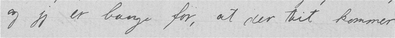og jeg er bange for, at der tit kommer
